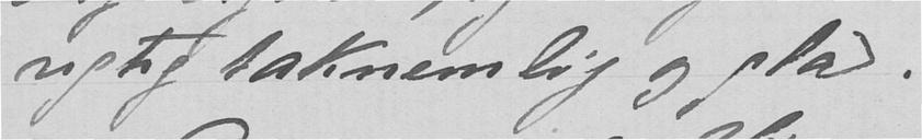rigtig taknemlig og glad.
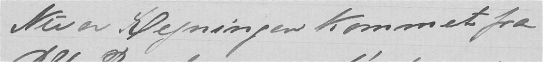Nu er Regningen kommet fra
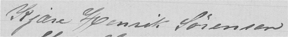Kjære Henrik Sørensen
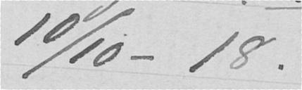10/10 - 18.
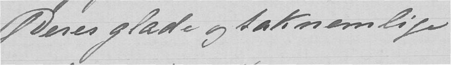Deres glade og taknemlige
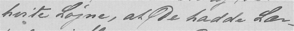hvite Løgne, at De hadde Lær-
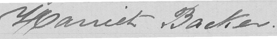Harriet Backer.
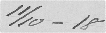11/10 - 18
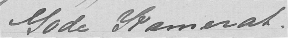Gode Kamerat.
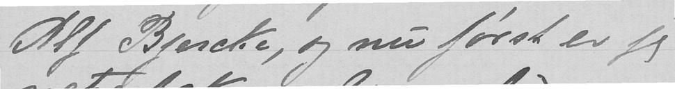Alf Bjercke, og nu først er jeg
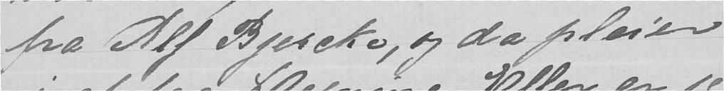fra Alf Bjercke, og da pleier
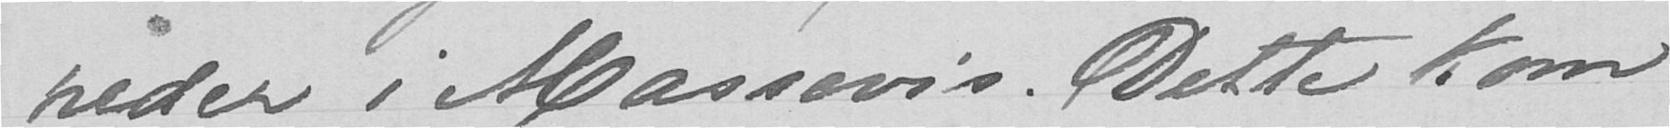reder i Massevis. Dette kom
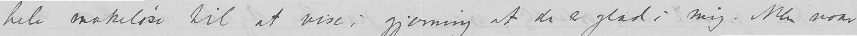hele makeløse til at vise i gjerning at de er glad i mig. Men naar
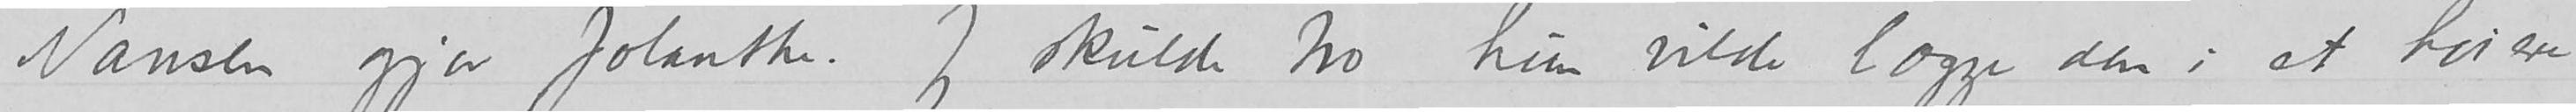Nansen gjør Jolanthe. Jeg skulde tro hun vilde logge den i et høiere
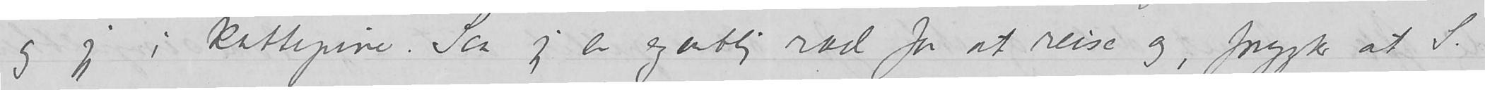og jeg i kattepine. Saa jeg er egentlig ræd for at reise og, frygter at S
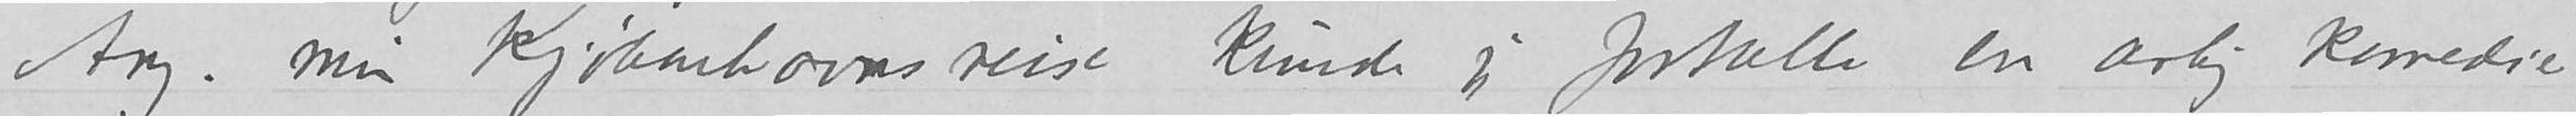Ang. min Kjøbenhavnsreise kunde jeg fortælle en artig komedie
